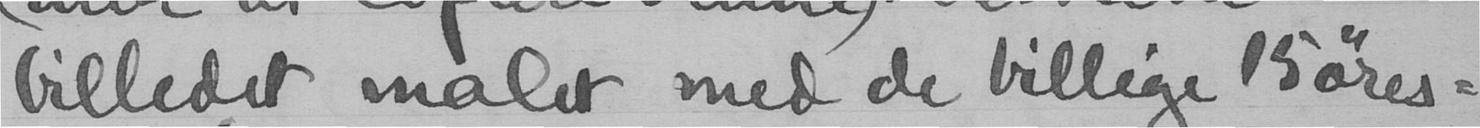billedet malet med de billige 15øres-
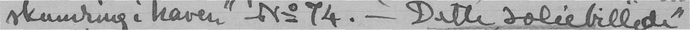skumring i haven" No 74. - Dette "soliebillede"
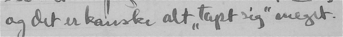og det er kanske alt "tapt sig" meget.
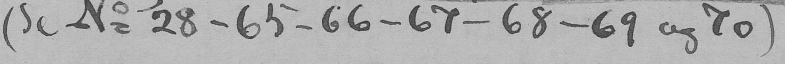(Se No 28 - 65 - 66 - 67 - 68 - 69 og 70)
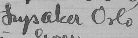Lysaker Oslo
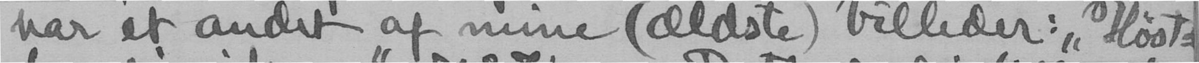har et andet af mine (ældste) billeder: "Høst-
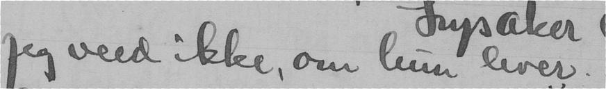Jeg veed ikke, om hun lever.
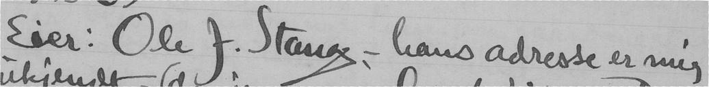Eier: Ole J. Stang, - hans adresse er mig
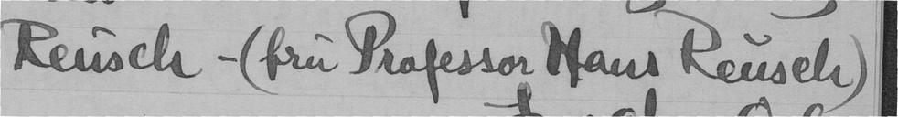Reusch - (fru Professor Hans Reusch)
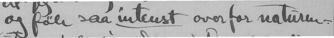og føle saa intenst overfor naturen-
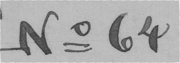No 64
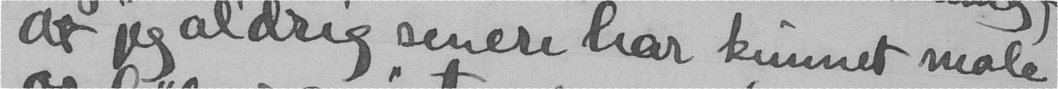at jeg aldrig senere har kunnet male
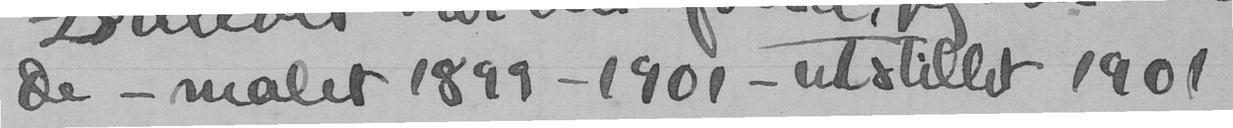de - malet 1899 - 1901 - udstillet 1901
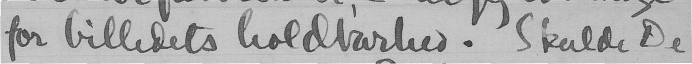for billedets holdbarhed. Skulde De
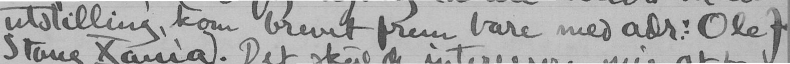utstilling, kom brevet frem bare med adr: Ole J.
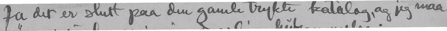Ja det er slutt paa den gamle trykte katalog, og jeg maa
